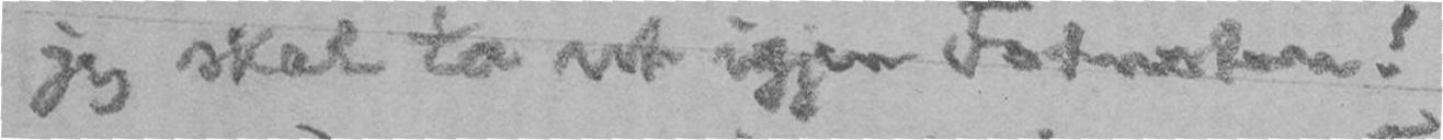jeg skal ta ut igjen Fotnoten!
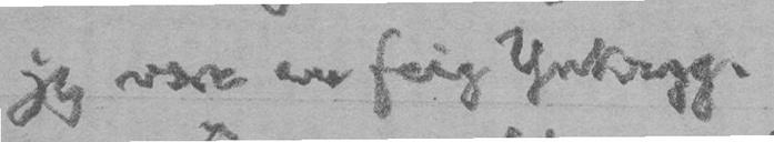jeg være er feig Ynkrygg.
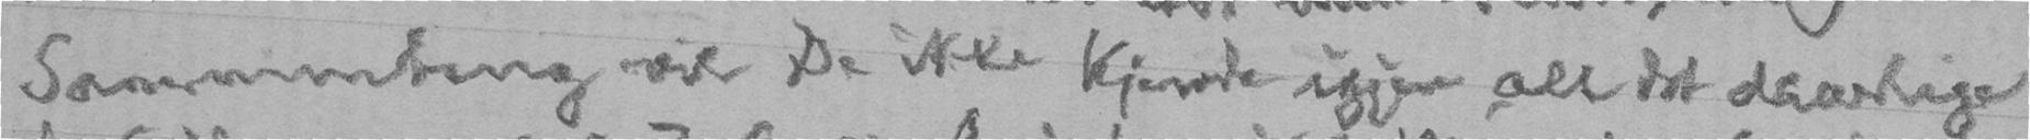Sammenheng vil De ikke kjende igjen alt det daarlige
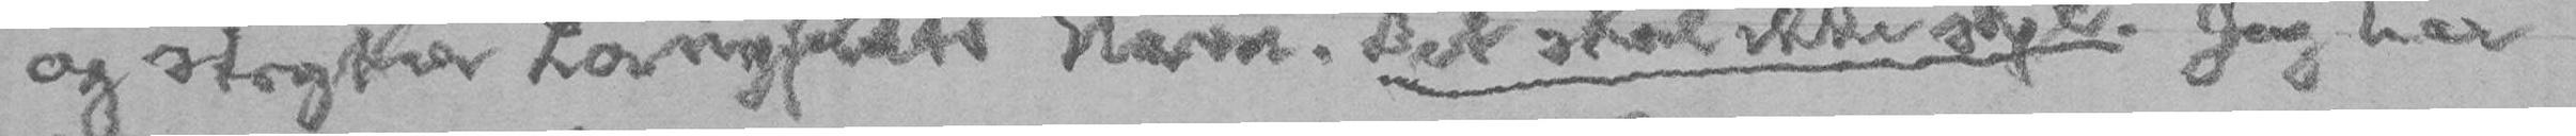og stryker Langfeldts Navn. Det skal ikke skje. Jeg har
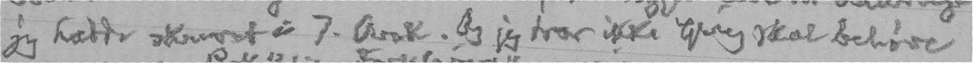jeg hadde skrevet i 7. Ark. Og jeg tror ikke Grieg skal behøve
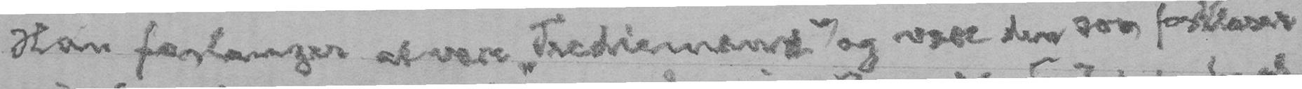Han forlanger at være "Trediemand" og være den som forklarer
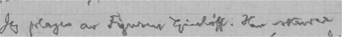Jeg plages av Figuren Gierløff. Han skriver
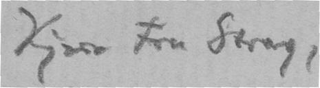Kjære Fru Stray,
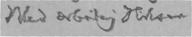Med ærbødig Hilsen
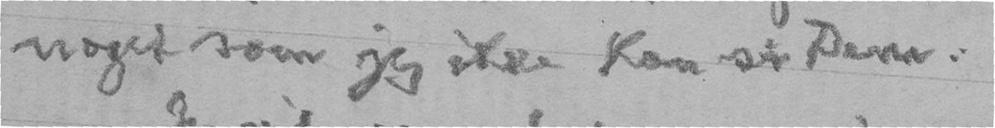noget som jeg ikke kan si Dem.
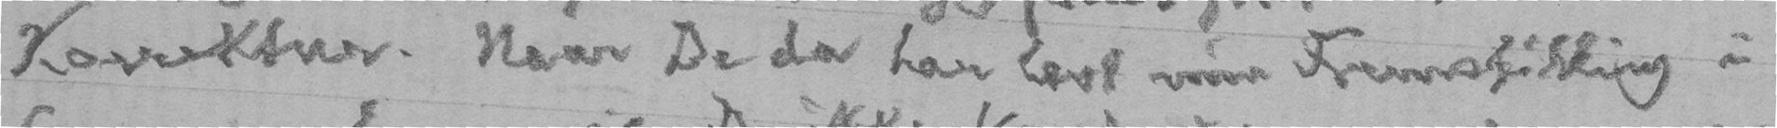Korrektur. Naar De da har læst min Fremstilling i
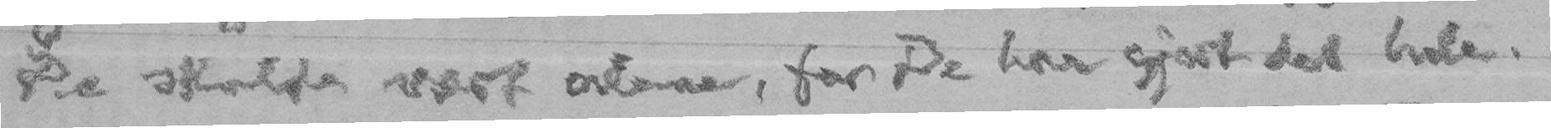De skulde vært alene, for De har gjort det hele.
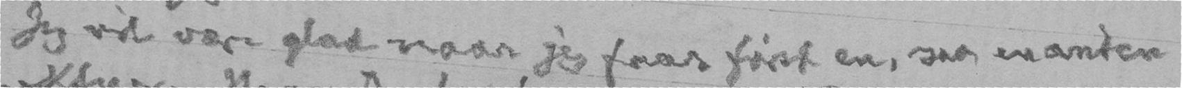Jeg vil være glad naar jeg faar først en, saa en anden
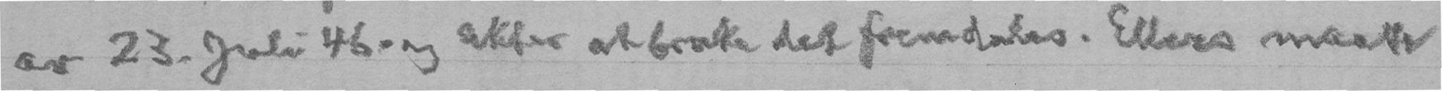av 23. Juli 46. og akter at bruke det fremdeles. Ellers maatte
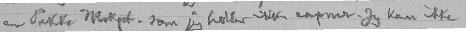en Pakke Mskpt. som jeg heller ikke aapner. Jeg kan ikke
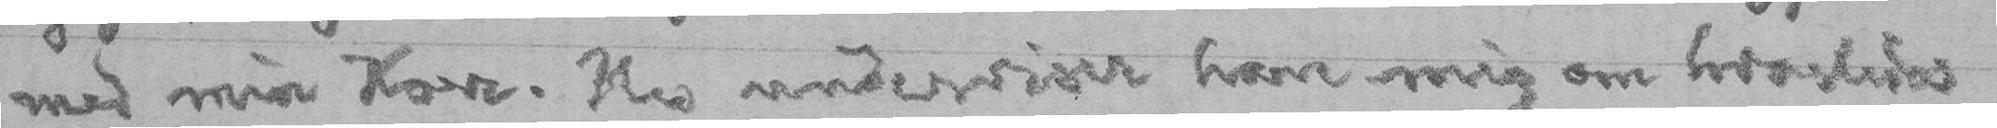med min Korr. Nu underviser han mig om hvorledes
